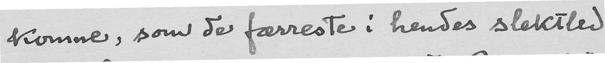komne, som de færreste i hendes slektled
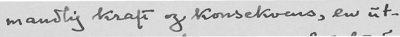mandlig kraft og konsekvens, en ut-
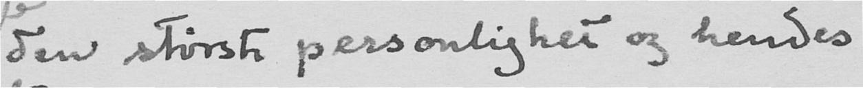den største personlighet og hendes
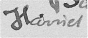Harriet
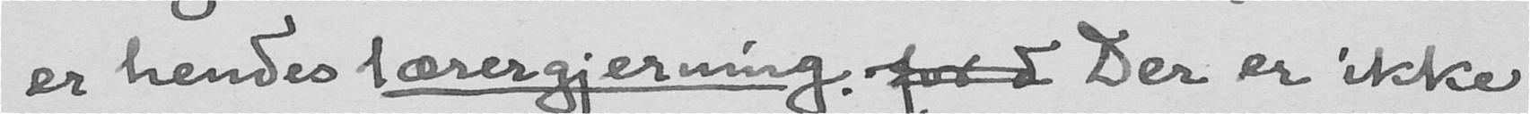er hendes lærergjerning. for d Der er ikke
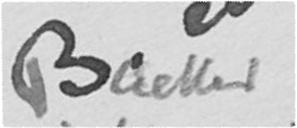Backer
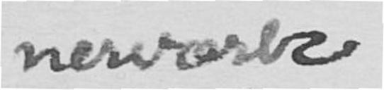nerværk
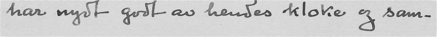har nydt godt av hendes kloke og sam-
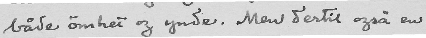både ømhet og ynde. Men dertil også en
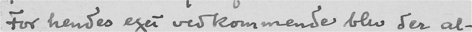For hendes eget vedkommende blev der al-
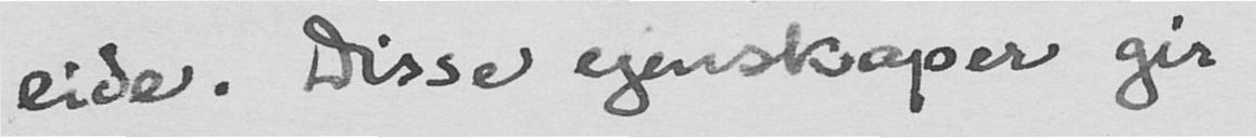eide. Disse egenskaper gir
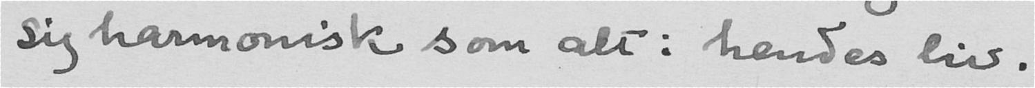sig harmonisk som alt i hendes liv.
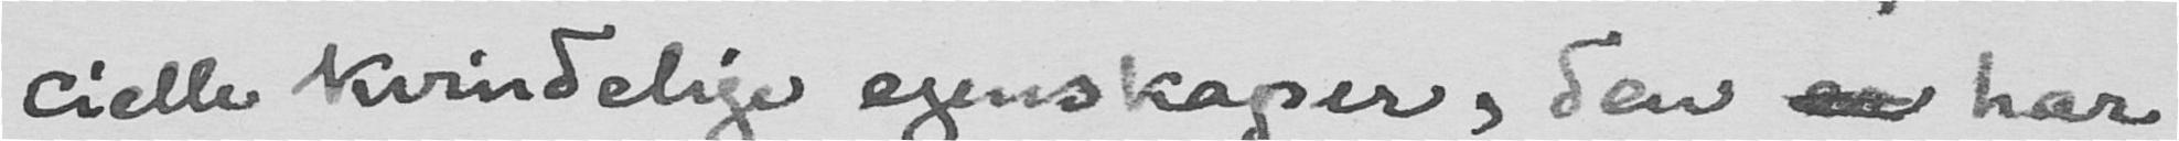cielle kvindelige egenskaper, den er har
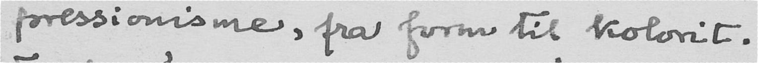pressionisme, fra form til kolorit.
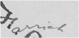Harriet
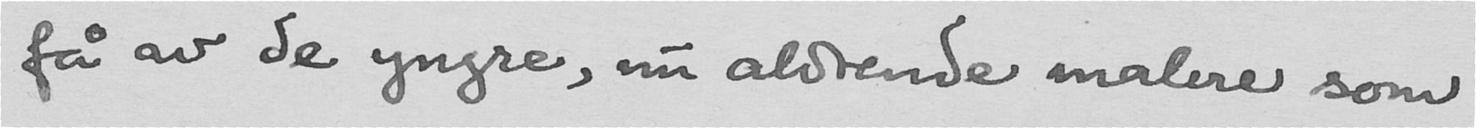få av de yngre, nu aldrende malere som
